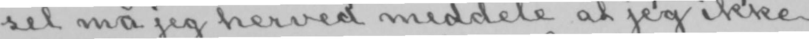sel må jeg herved meddele at jeg ikke
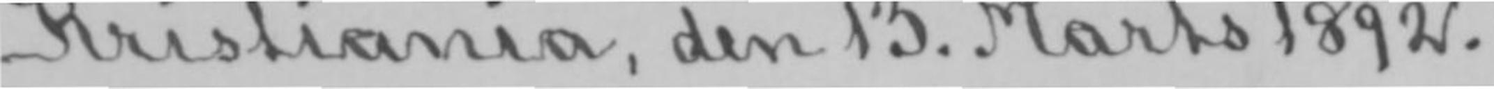Kristiania, den 13. Marts 1892.
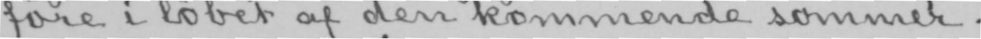føre i løbet af den kommende sommer.
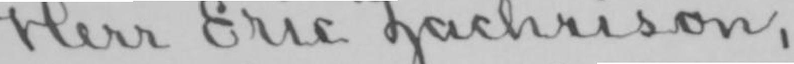Herr Eric Zachrison,
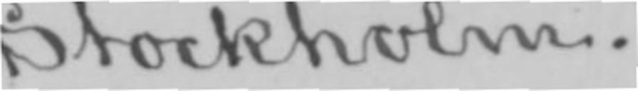Stockholm.
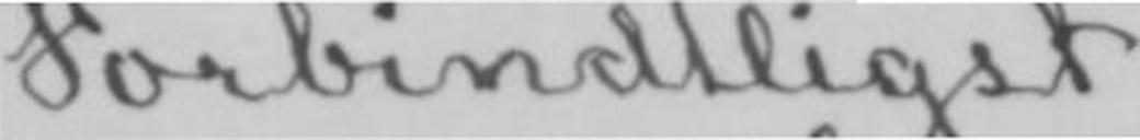Forbindtligst
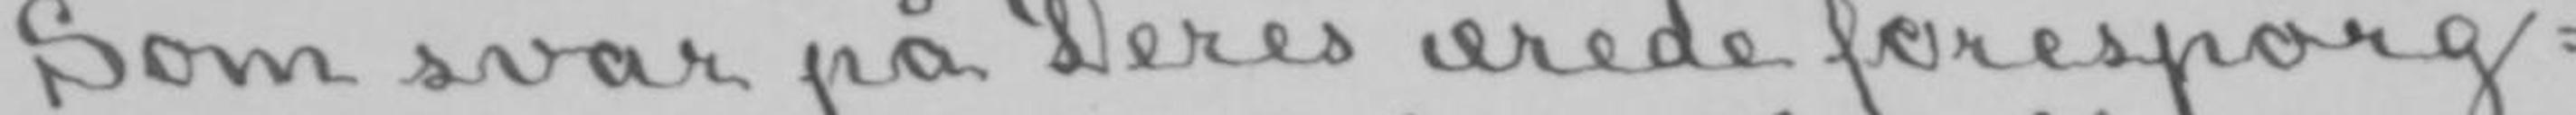Som svar på Deres ærede forespørg-
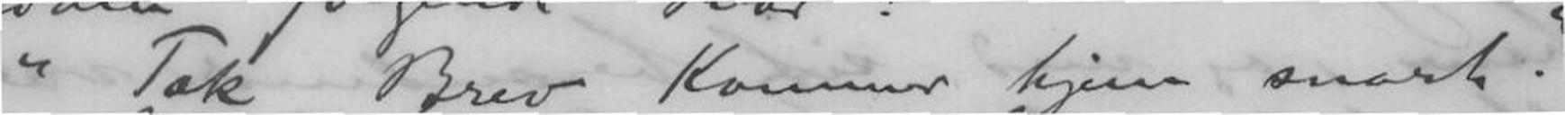"Tak Brev kommer hjem snart."
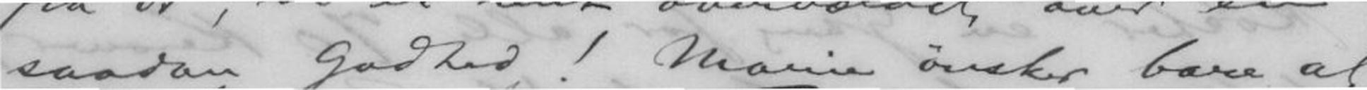saadan godhed! Marie ønsker bare at
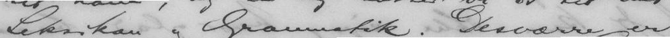Leksikon og Grammatik. Desværre er
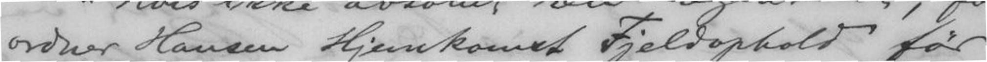ordner Hansen Hjemkomst Fjeldophold før
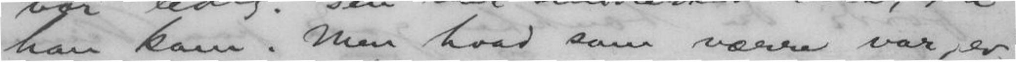han kom. Men hvad som værre var, er,
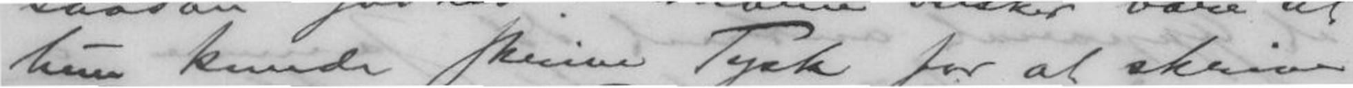hun kunde skrive Tysk for at skrive
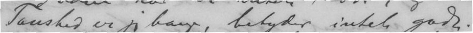Taushed, er jeg bange, betyder intet godt.
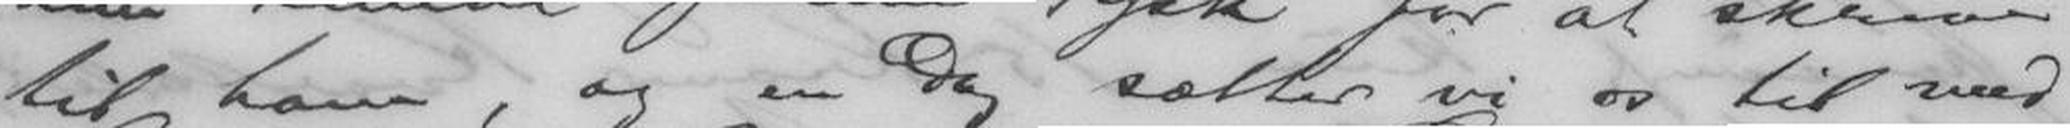til ham, og en Dag sætter vi os til med
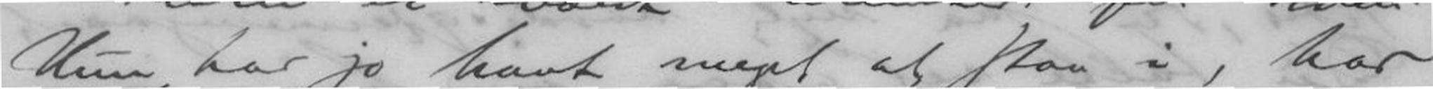Hun har jo havt meget at staa i, har
